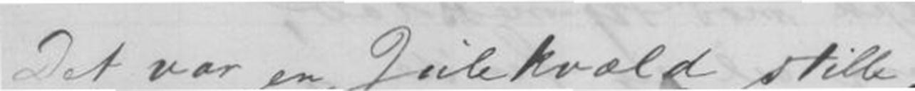Det var en Julekveld stille,
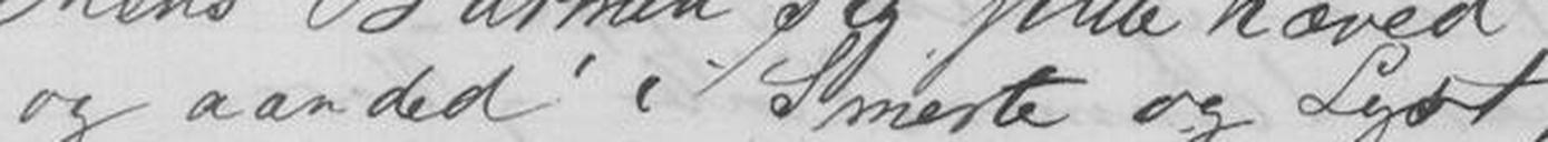og aanded i Smerte og Lyst,
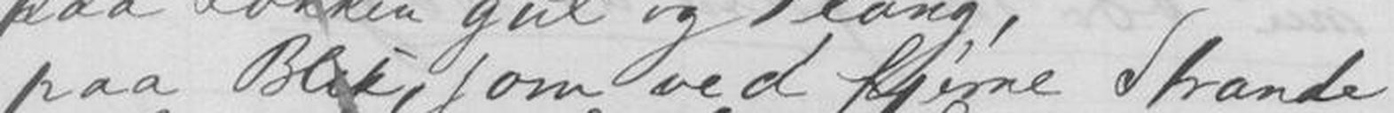paa Blik, som ved fjerne Strande
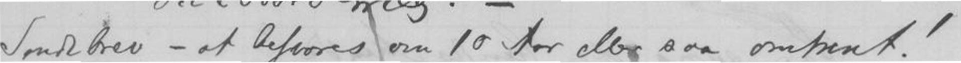Sandbrev - at besvares om 10 Aar eller saa omtrent!
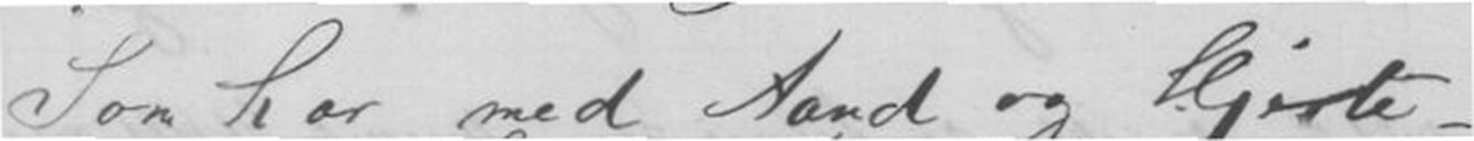Som har med Aand og Hjerte -
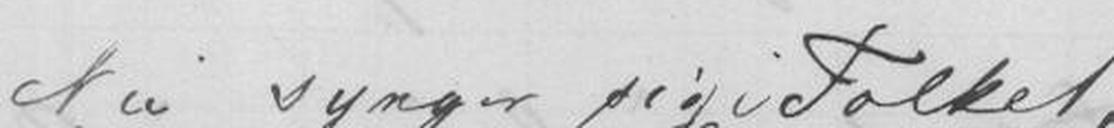Nu synger sig i Folket,
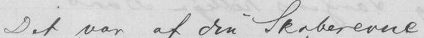Det var af din Skaberevne
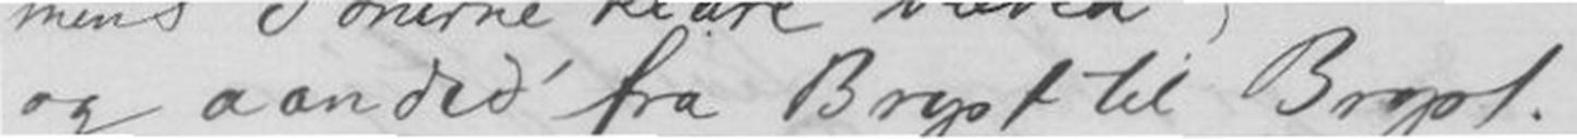og aanded fra Bryst til Bryst.
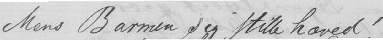Mens Barmen sig stille hæved!
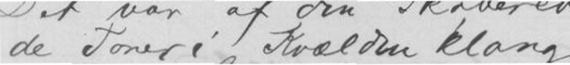de Toner i Kvælden klang,
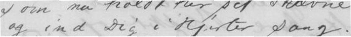og ind Dig i Hjerter sang.
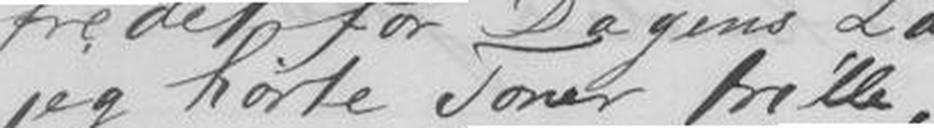jeg hørte Toner trille,
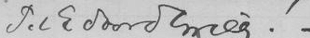Til Edvard Grieg! -
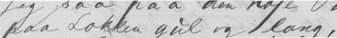paa Lokken gul og lang,
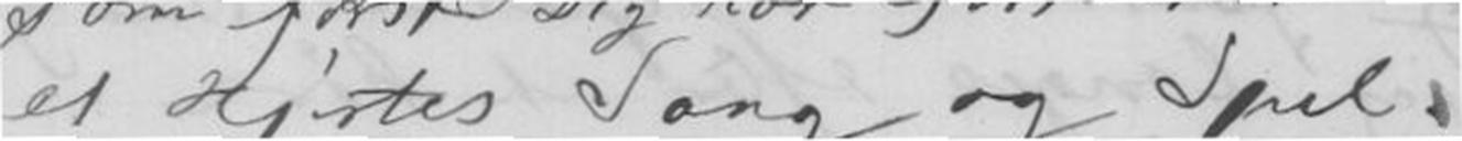et Hjertes Sang og Spil.
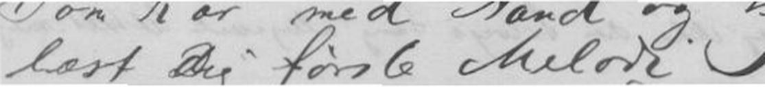læst Dig første Melodi
